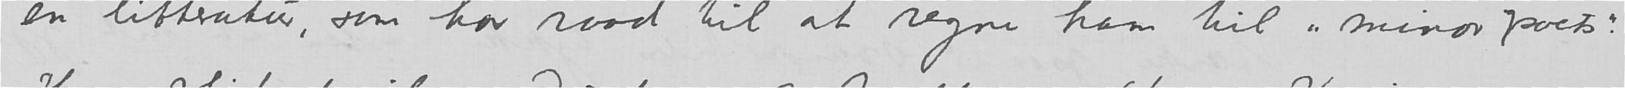en litteratur, som har raad til at regne ham til «minor poets»
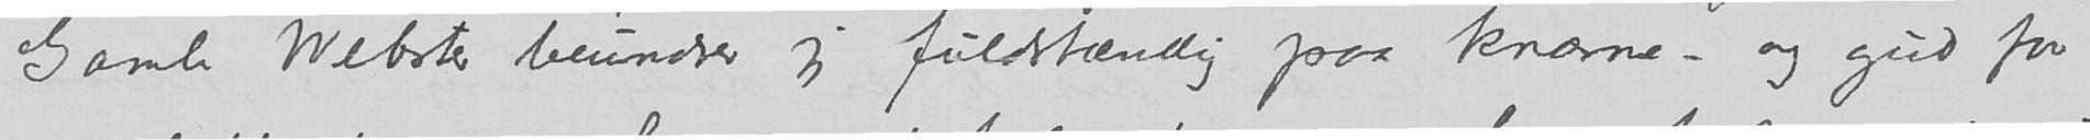Gamle Webster beundrer jeg fuldstændig paa knærne – og gud for
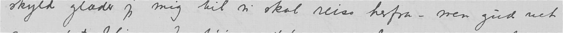skyld glæder jeg mig til vi skal reise herfra – men gud vet
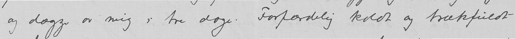og dægge av mig i tre dage. Forfærdelig koldt og trækfuldt
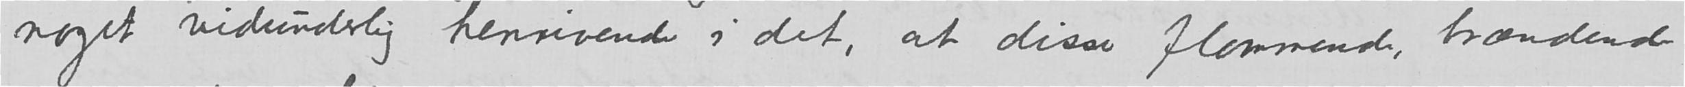noget vidunderlig henrivende i det, at disse flammende, brændende
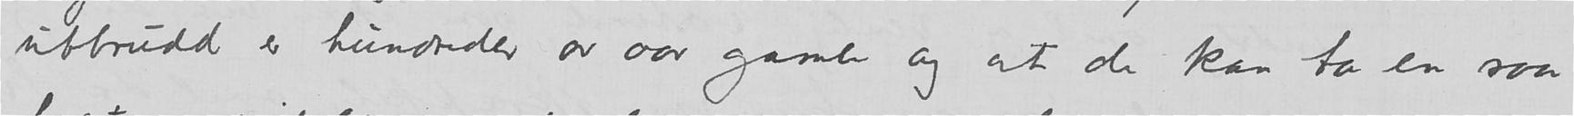utbrudd er hundreder av aar gamle og at de kan ta en saa
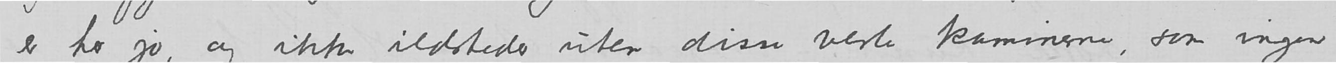er her jo, og ikke ildsteder uten disse vesle kaminerne, som ingen
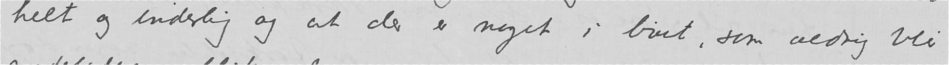helt og inderlig og at der er noget i livet, som aldrig blir
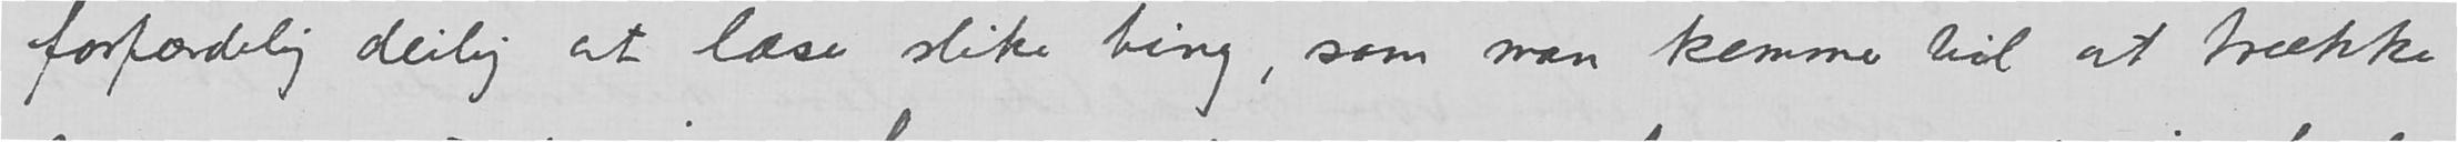forfærdelig deilig at læse slike ting, som man kommer til at trække
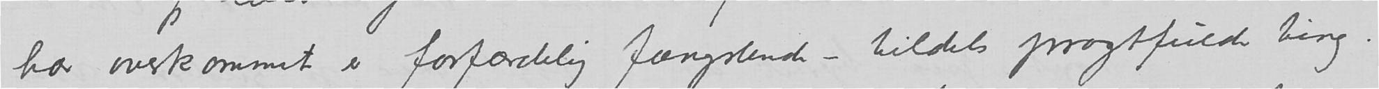har overkommet er forfærdelig fængslende – tildels pragtfulde ting.
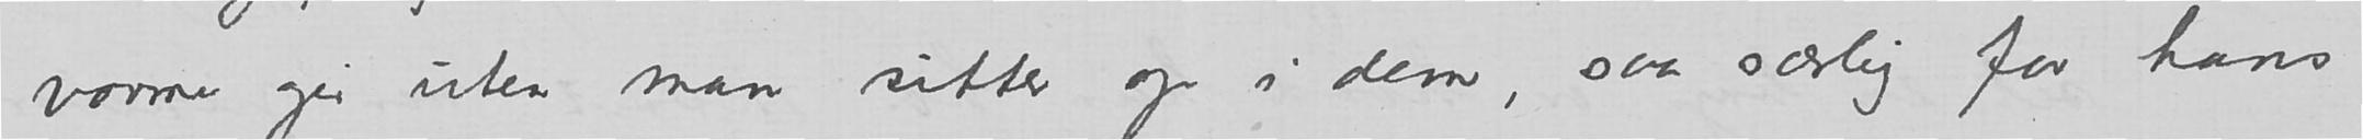varme gir uten man sitter op i dem, saa særlig for hans
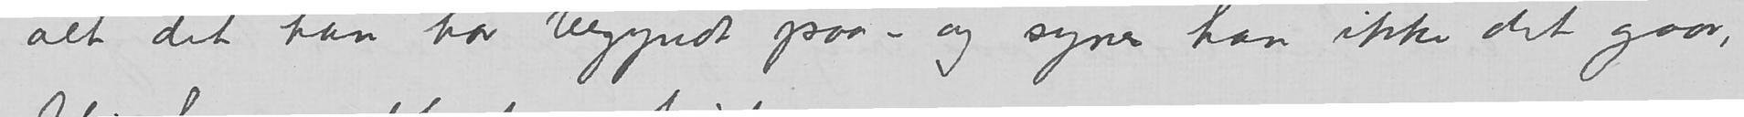alt det han har begyndt paa – og synes han ikke det gaar,
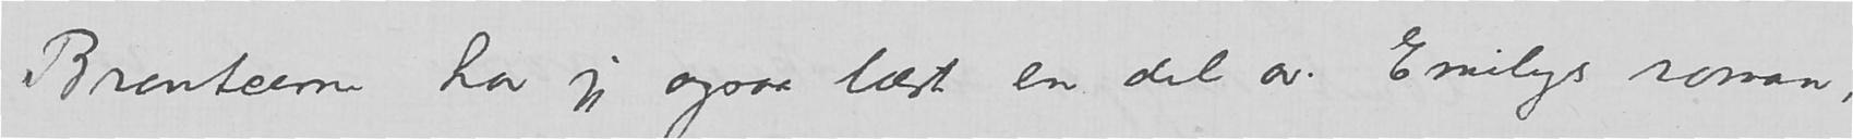Bronteerne har jeg ogsaa læst en del av. Emilys roman,
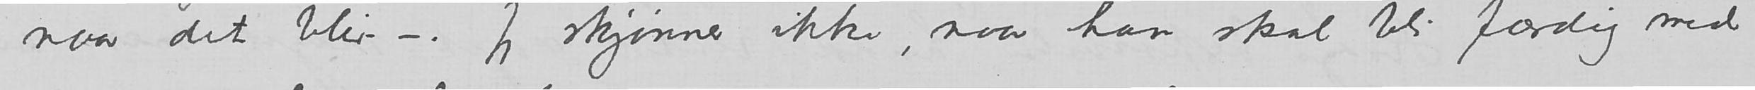naar det blir –. Jeg skjønner ikke, naar han skal bli færdig med
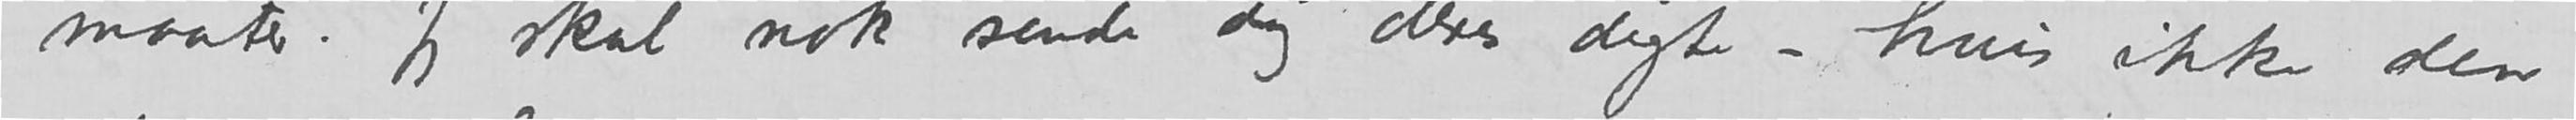maater. Jeg skal nok sende dig disse digte – hvis ikke den
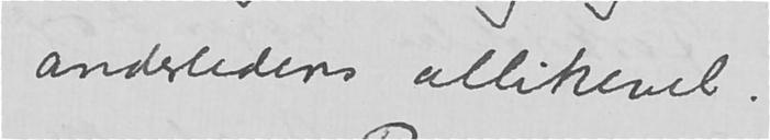anderledens allikevel.
